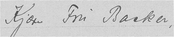Kjære Fru Backer,
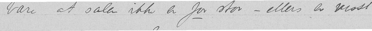bare at salen ikke er for stor – ellers er visst
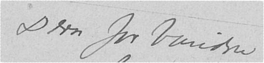Deres forbundne
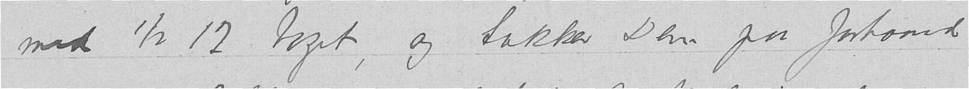med ½ 12 toget, og takker Dem paa forhaand
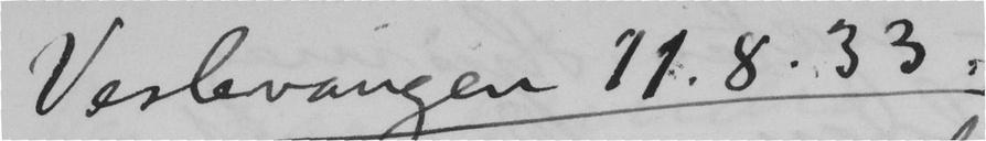Veslevangen 11.8.33.
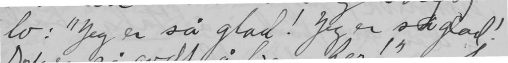lo: "Jeg er så glad! Jeg er så glad.
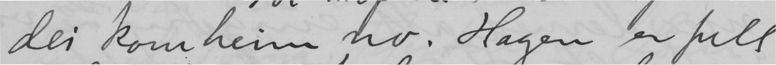dei kom heim no. Hagen er full
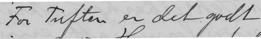For Tuften er det godt
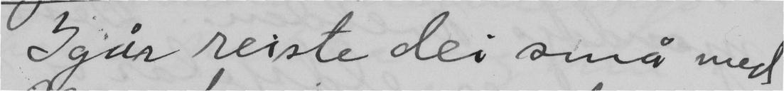Igår reiste dei små med
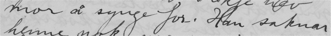mor å synge for. Han saknar
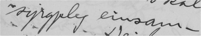syrgjeleg einsam –
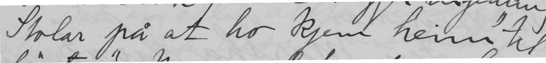stolar på at ho kjem heim til
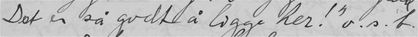Det er så godt å ligge her" o.s.b.
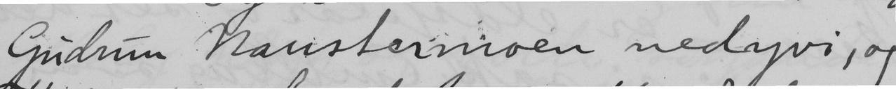Gudrun Naustermoen nedyvi, og
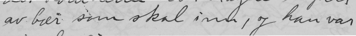av bær som skal inn, og han var
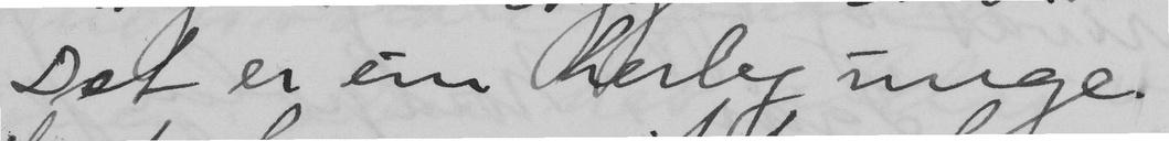Det er ein herleg unge.
